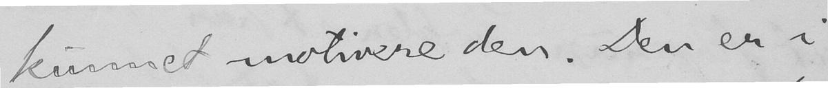kunnet motivere den. Den er i
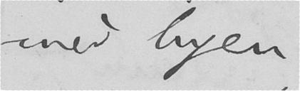med byen.
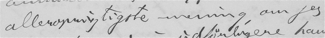alleroprigtigste mening, om jeg
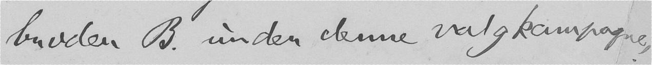broder B. under denne valgkampagne!
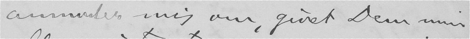anmoder mig om, givet Dem min
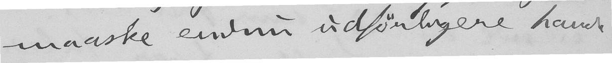maaske endnu udførligere havde
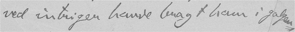ved intrigen havde bragt ham i galgen,
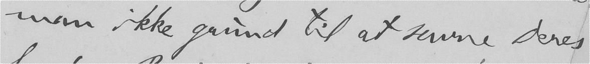man ikke grund til at savne Deres
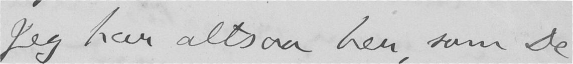Jeg har altsaa her, som De
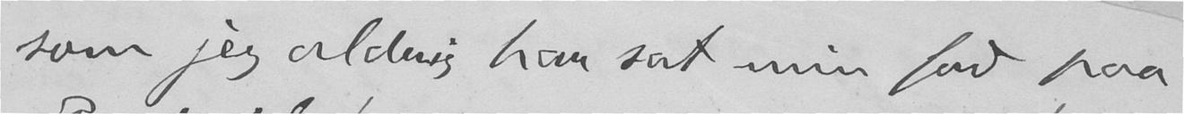som jeg aldrig har sat min fod paa
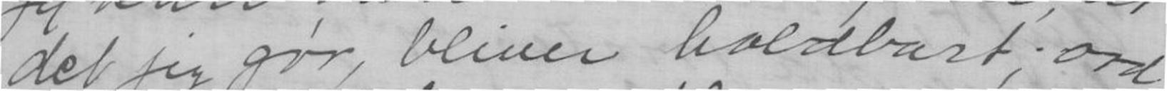det jeg gör, bliver holdbart; ord-
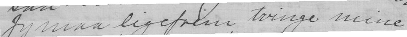Jeg maa ligefrem tvinge mine
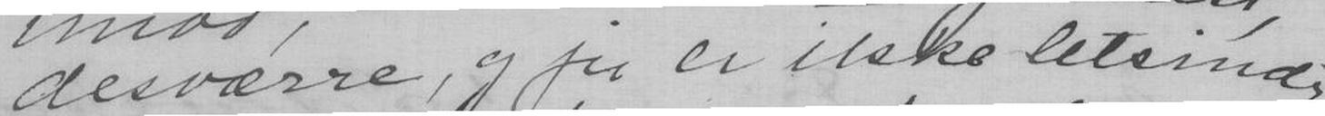dessværre, og jeg er ikke letsindig
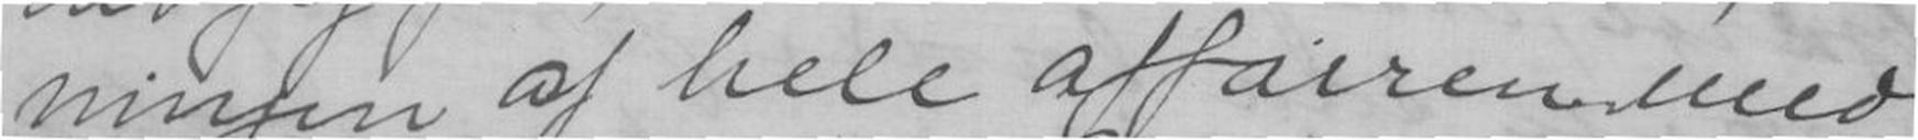ningen af hele affairen med
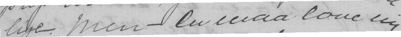lige. Men - Du maa love mig,
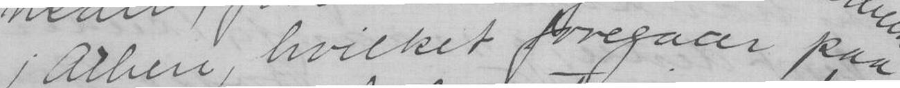i Athen, hvilket foregaar paa
